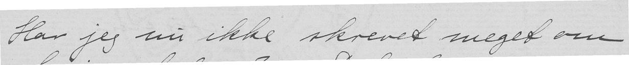Har jeg nu ikke skrevet meget om
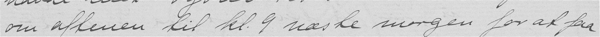om aftenen til kl. 9 næste morgen for at faa
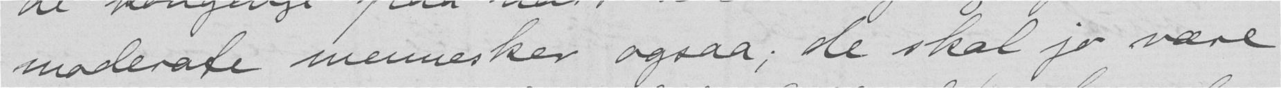moderate mennesker ogsaa; de skal jo være
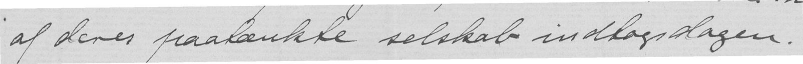af deres paatænkte selskab indtogsdagen.
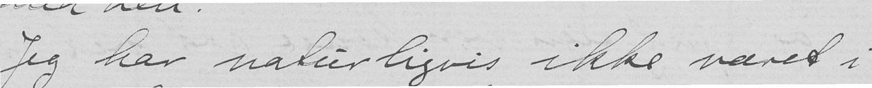Jeg har naturligvis ikke været i
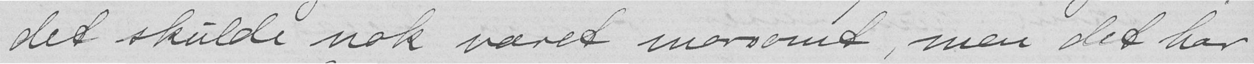det skulde nok været morsomt, men det har
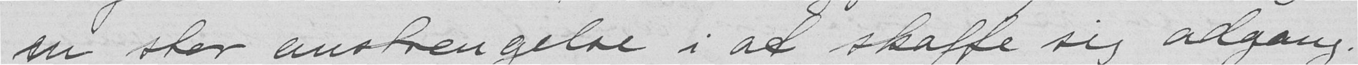en stor anstrengelse i at skaffe sig adgang.
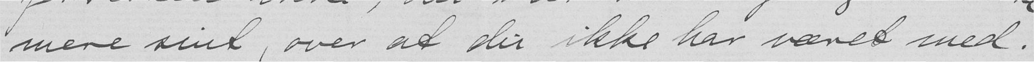mere sint, over at du ikke har været med.
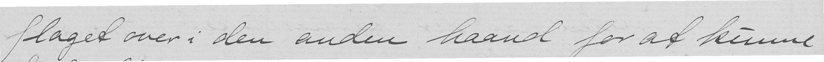flaget over i den anden haand for at kunne
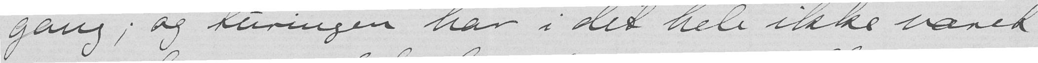gang; og turingen har i det hele ikke været
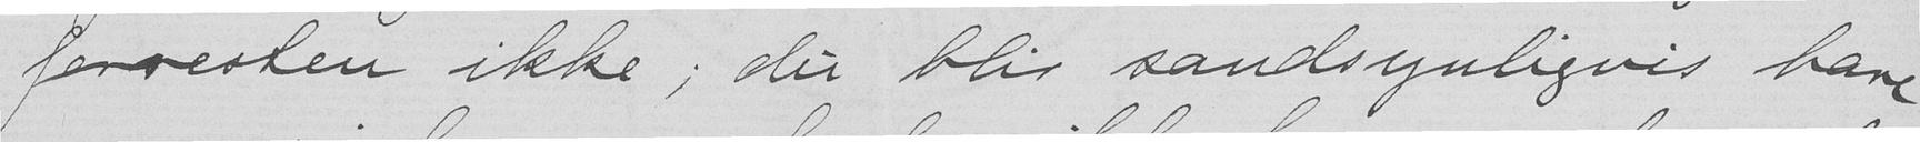forresten ikke; du blir sandsynligvis bare
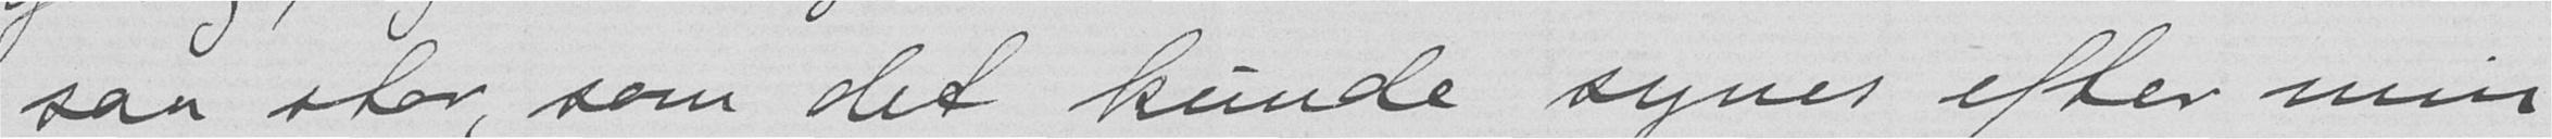saa stor, som det kunde synes efter min
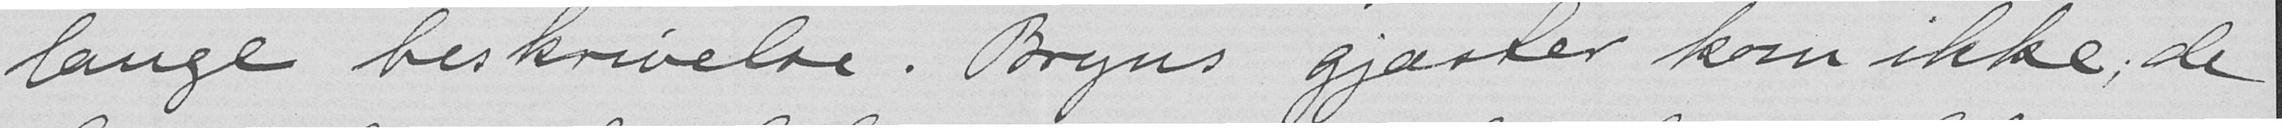lange beskrivelse. Bryns gjæster kom ikke; de
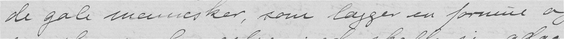de gale mennesker, som lægger en formue og
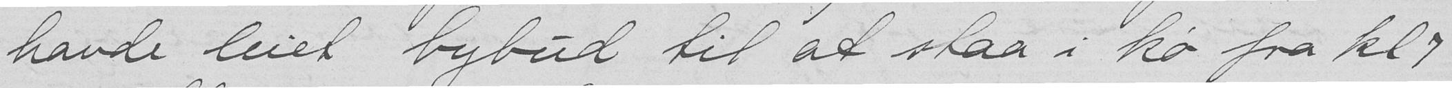havde leiet bybud til at staa i kø fra kl 7
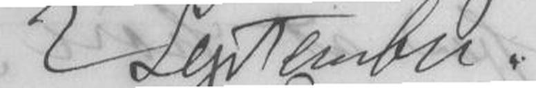2 September.
